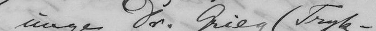unge Dr. Grieg (Tryk-
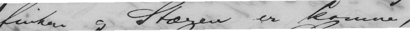finken og Stæren er komne,
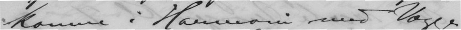komme i Harmoni med Vægge og
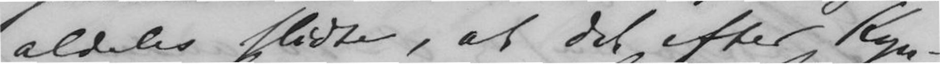aldeles slidte, at det efter Kyn-
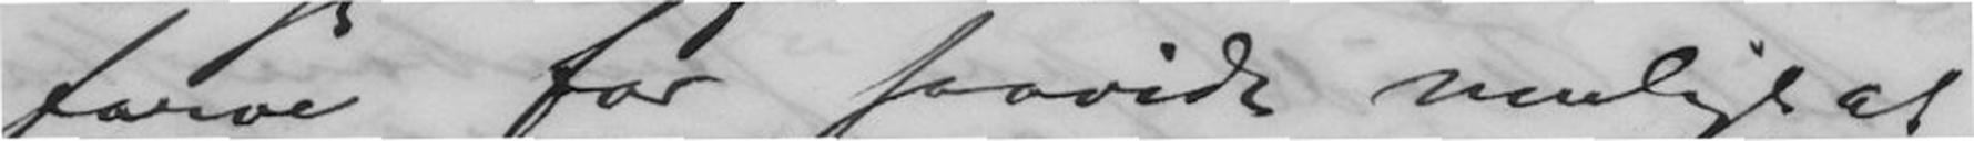farve for saavidt muligt at
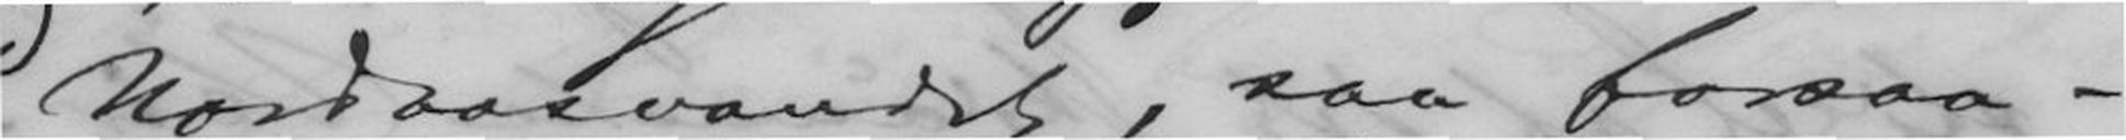Nordaasvandet, saa forsaa-
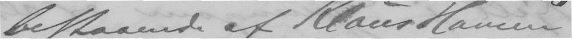bestaaende af Klaus Hansen,
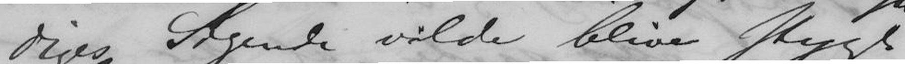diges Sigende vilde blive stygt
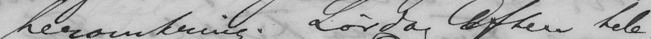heromkring. Lørdag Aften tele
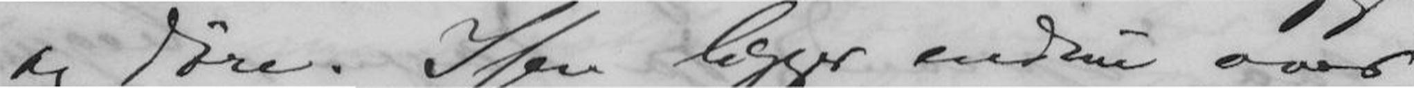Døre. Isen ligger endnu over
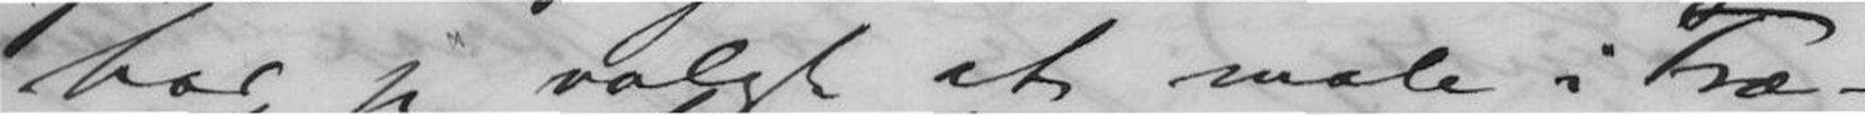har jeg valgt at male i Træ-
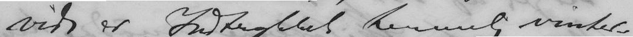vidt er Indtrykket temmelig vinter-
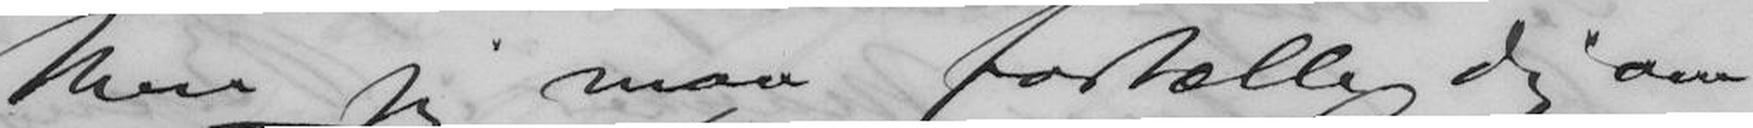Men jeg maa fortælle dig om
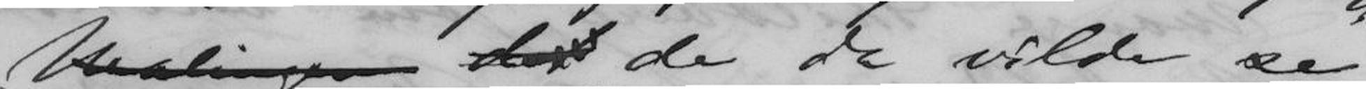Malingen da/det de da vilde se
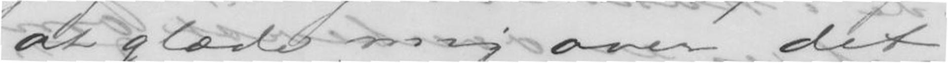at glæde mig over det
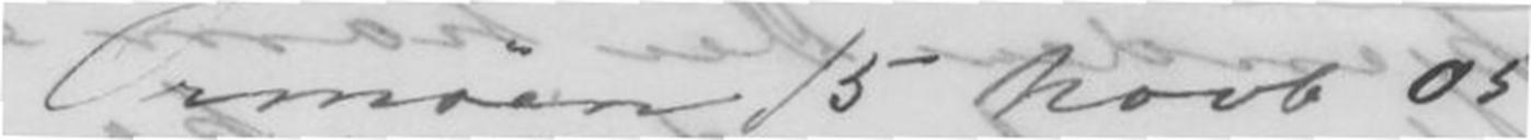Ormøen 15 Novb. 05.
